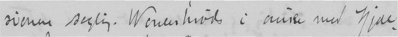sionen saglig. Werenskiold i avisen med Hjal-
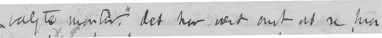«valgte montør», det har vært ondt at se, hvor
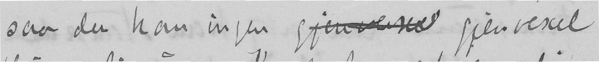saa der kan ingen gjenvexere gjenvexel
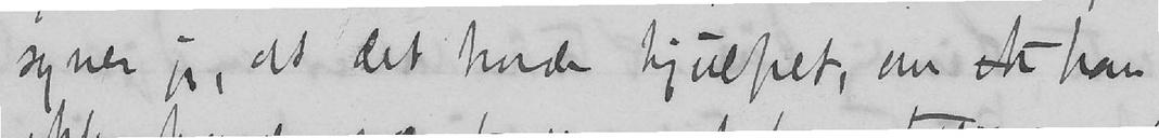synes jeg, at det kunde hjulpet, om ik han
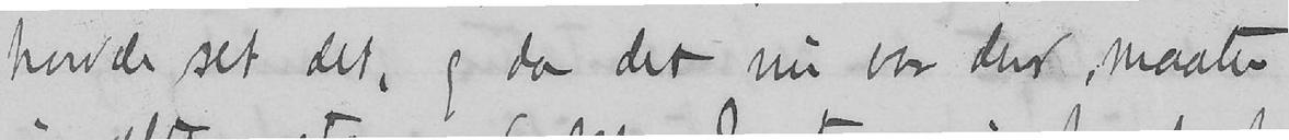havde set det, og da det nu var der, maatte
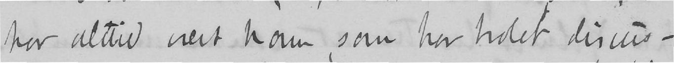har altid vært ham, som har holdt discus-
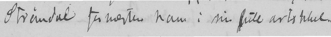Strømdal fornægter ham i sin fule artikkel.
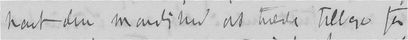havt den mandighed at træde tilbage for
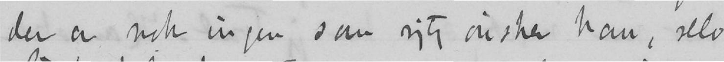der er nok ingen som rigtig ønsker ham, selv
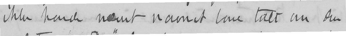ikke havde nævnt navnet bare talt om den
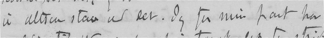vi altsaa staa ud det. Jeg for min part har
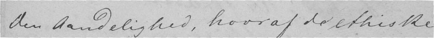Den Aandelighed, hvoraf de ethiske
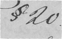§ 20.
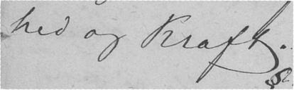hed og Kraft.
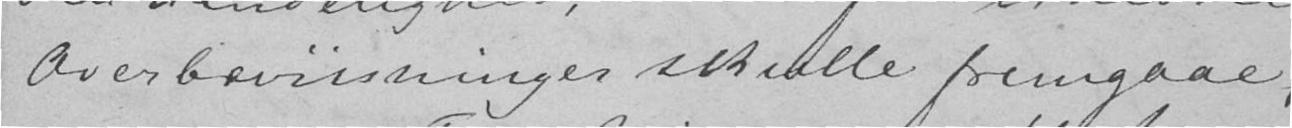Overbevisninger skulle fremgaae,
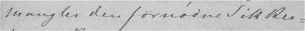mangler den fornødne Sikker-
